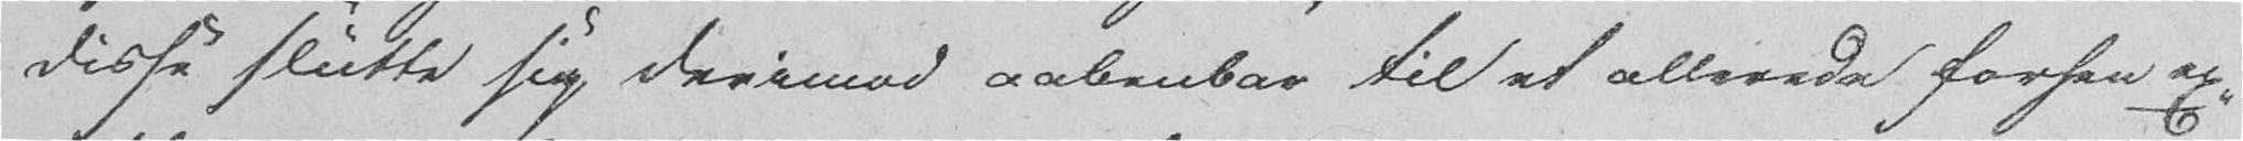disse slutte sig derimod aabenbar til et allerede forhen ex-
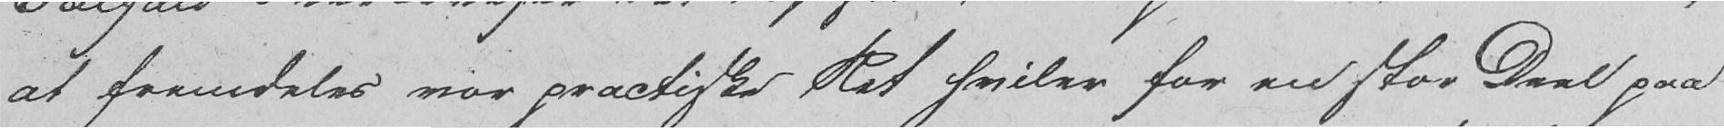at fremdeles vor practiske Ret hviler for en stor Deel paa
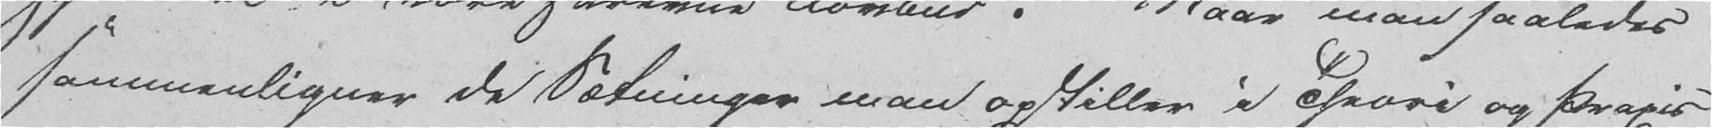sammenligner de Sætninger man opstiller i Theori og Praxis
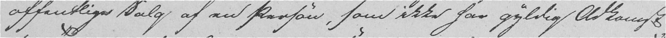offentlige Salg af en Person, som ikke har gyldig Adkomst
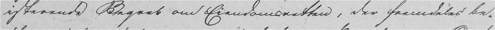isterende Begreb om Eiendomsretten, der fremdeles be-
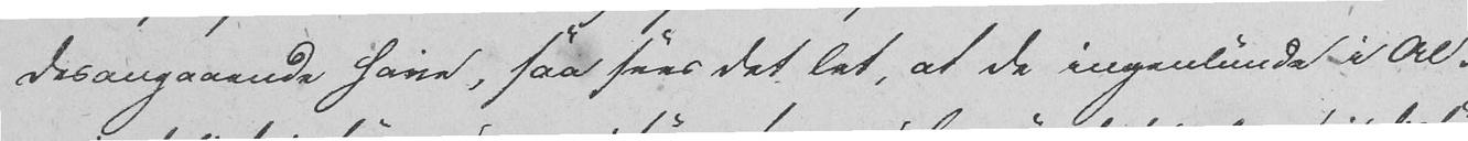desangaaende have, saa sees det let, at de ingenlunde i Al-
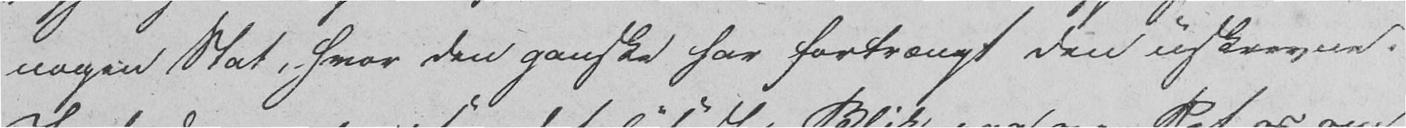nogen Stat, hvor den ganske har fortrængt den uskrevne.
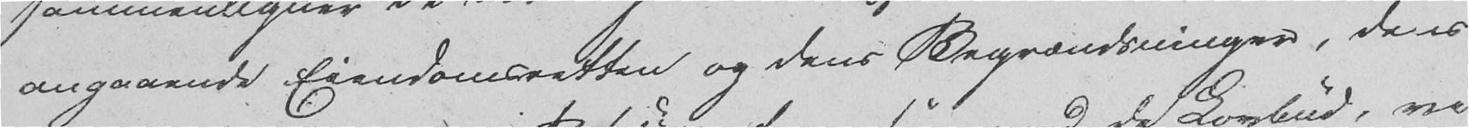angaaende Eiendomsretten og dens Begrændsninger, dens
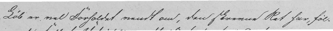Løb er vel Forholdet vendt om, den skrevne Ret har, føl-
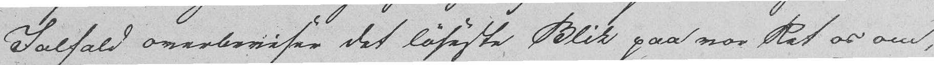I alfald overbeviser det løseste Blik paa vor Ret os om,
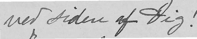ved siden af Dig!
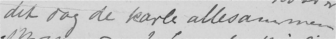det dog de karle allesammen
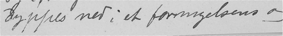dyppes ned i et fornyelsens og
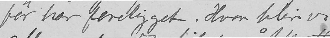før har foreligget. Hvor blir vi
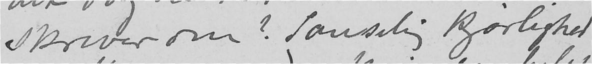skriver om? Sanselig kjærlighed
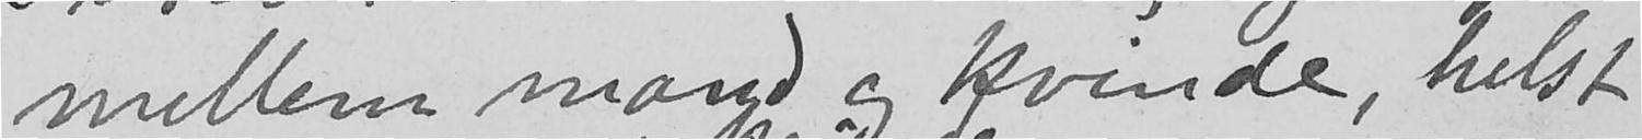mellem mand og kvinde, helst
